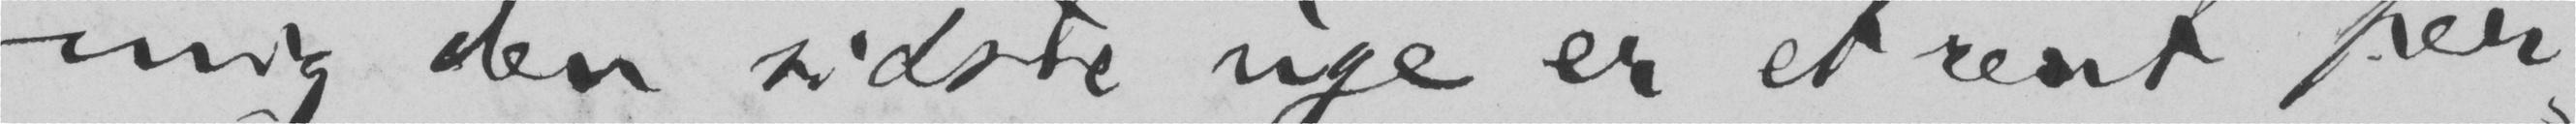mig den sidste uge er et rent per-
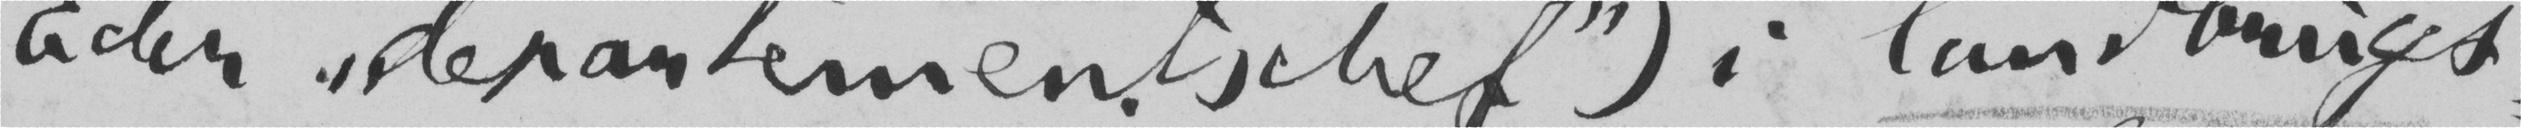Eder «departementschef») i landbrugs-
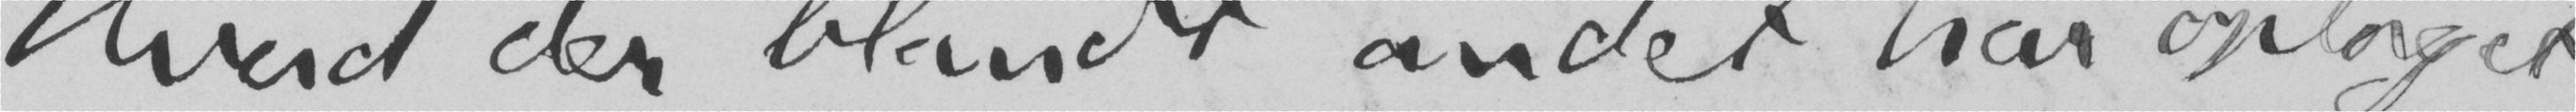Hvad der blandt andet har optaget
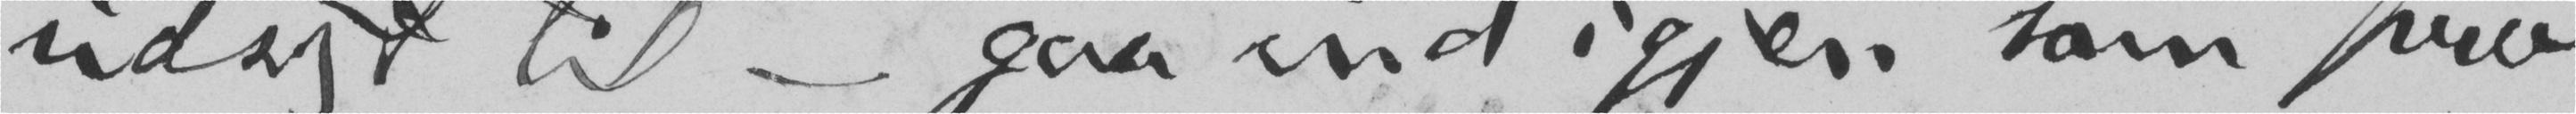udsigt til – gaa ind igjen som pro-
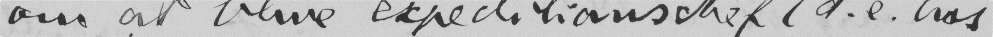om at blive expeditionschef (d.e. hos
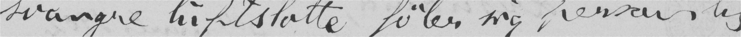svangre luftslotte, føler sig personlig
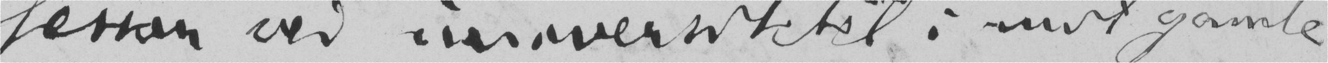fessor ved universitetet i mit gamle
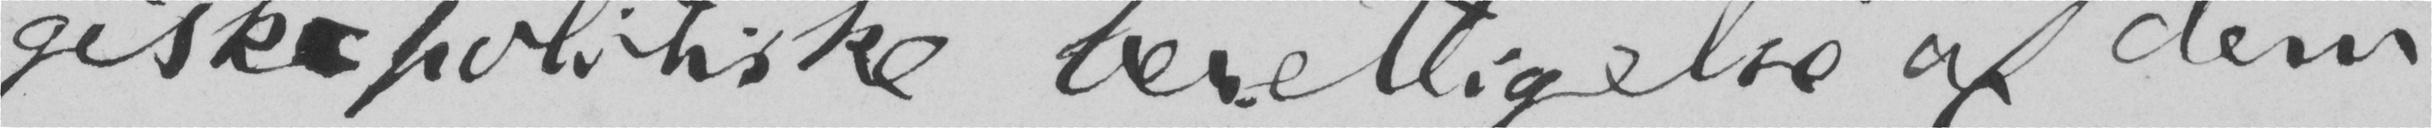giskepolitiske berettigelse af dem.
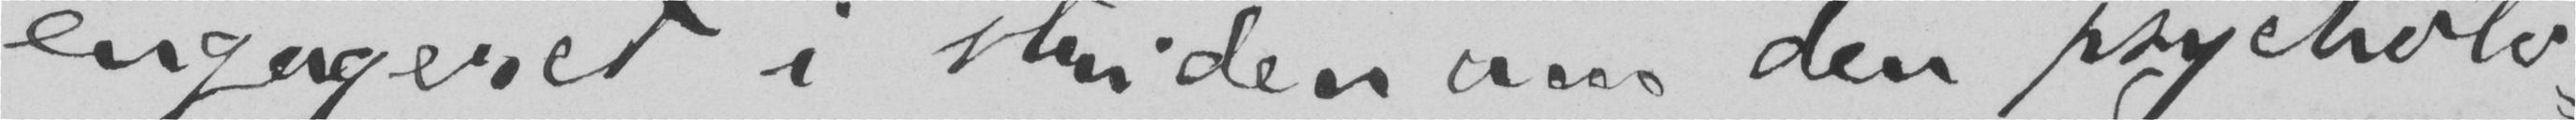engageret i striden om den psycholo-
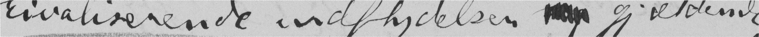rivaliserende indflydelser gjældende,
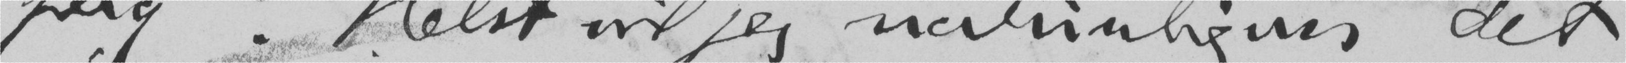fag? Helst vil jeg naturligvis det
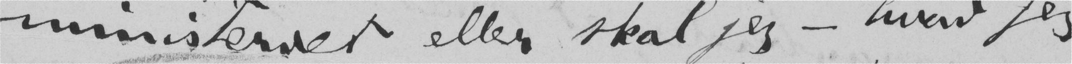ministeriet eller skal jeg – hvad jeg
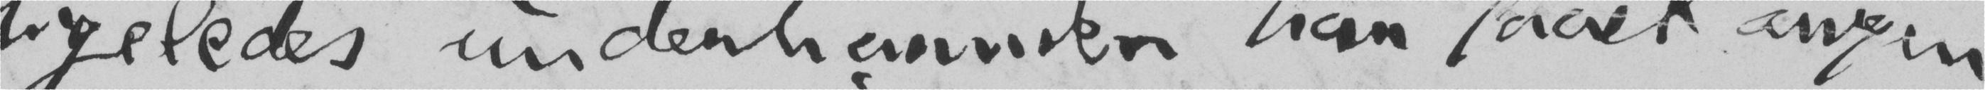ligeledes underhaanden har faaet angin
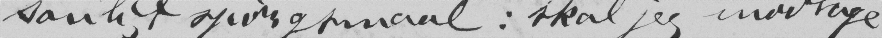sonligt spørgsmaal: skal jeg modtage
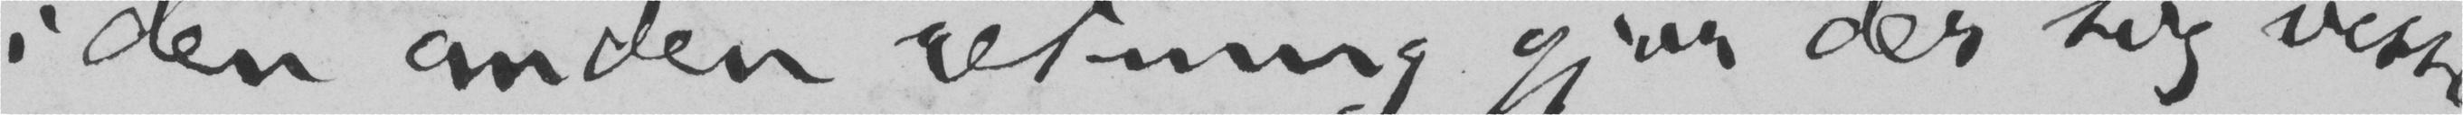i den anden retning gjør der sig visse
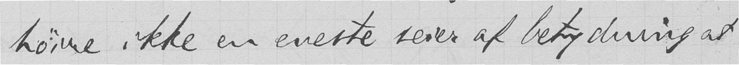høire ikke en eneste seier af betydning at
